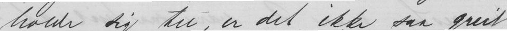at holde sig til, er det ikke saa greit
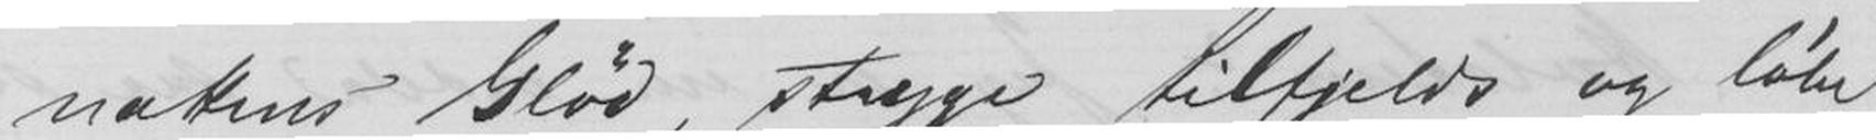nattens Glød, stryge tilfjelds og løbe
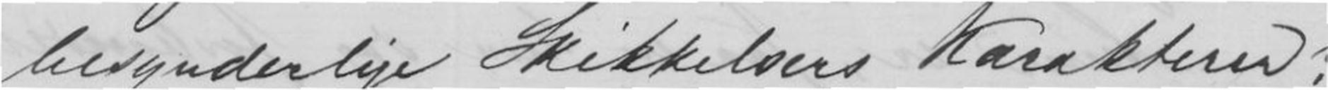besynderlige Skikkelsers Karakterer?
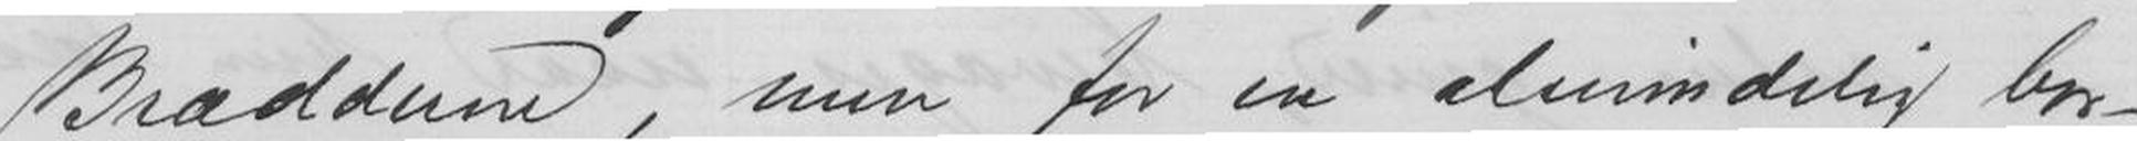Brædderne, men for en almindelig bor-
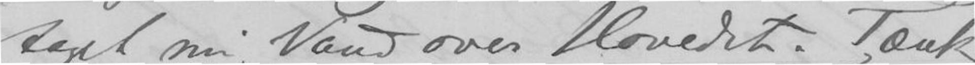taget mig Vand over Hovedet. Tænk
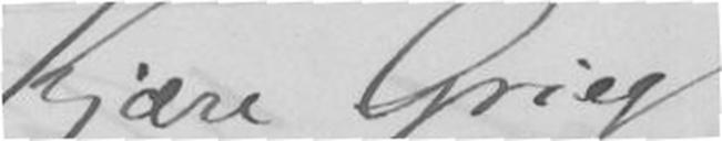Kjære Grieg!
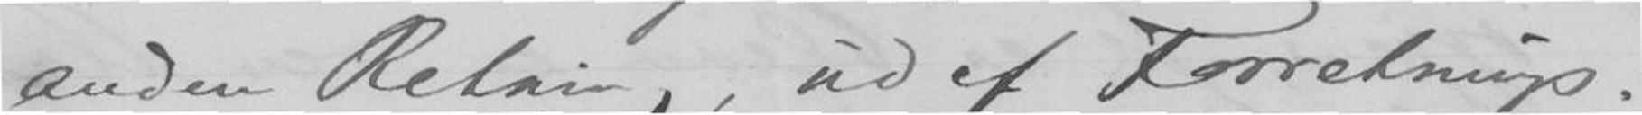anden Retning, ud af Forretnings-
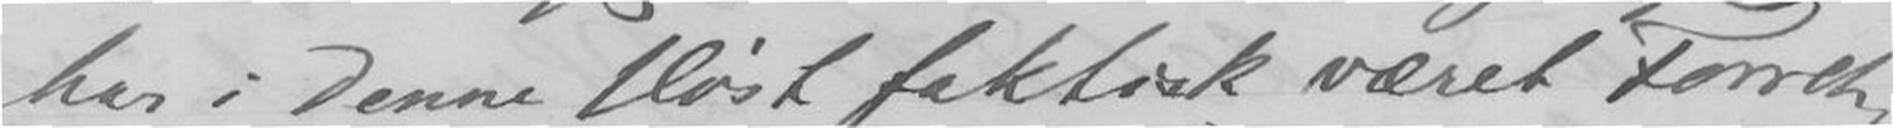i denne Høst faktisk været Forretnings-
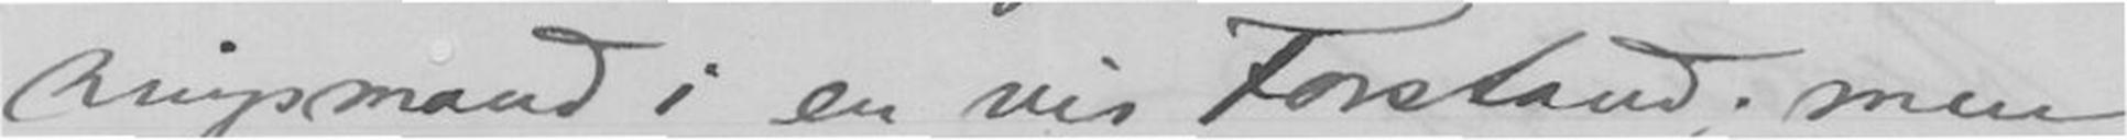mand i en vis Forstand; men
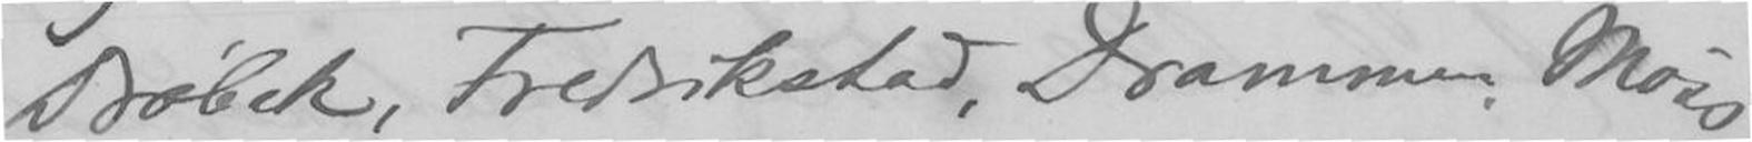Drøbak, Fredrikstad, Drammen, Moss
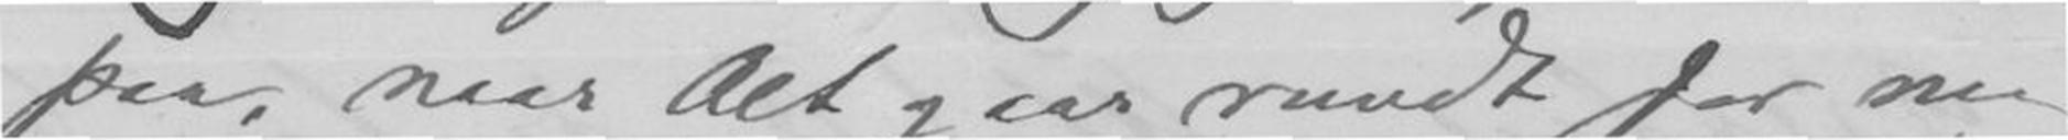paa, naar Alt daar rundt for mig
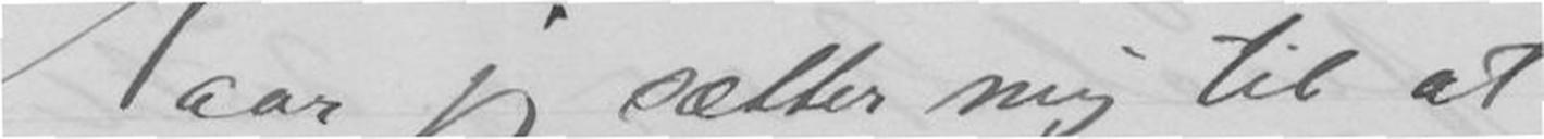Naar jeg sætter mig til at
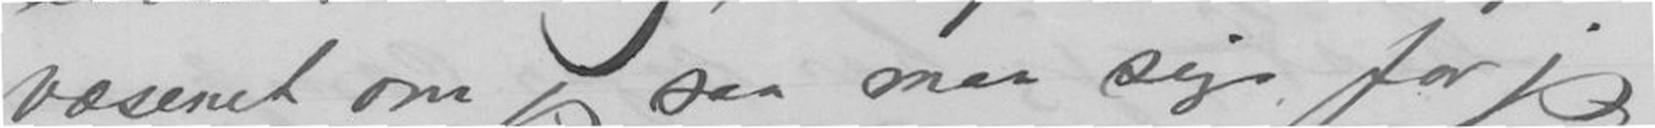væsenet om jeg saa maa sige for jeg har
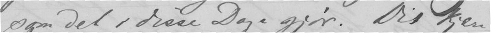som det i disse Dage gjør. Dit kjære
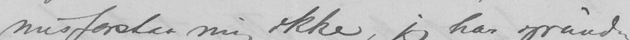misforstaa mig ikke, jeg har igrunden
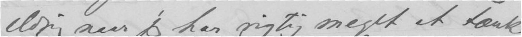aldrig naar jeg har rigtig meget at tænke
